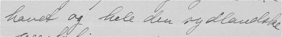havet og hele den sydlandske
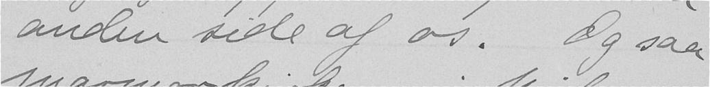anden side af os. Og saa
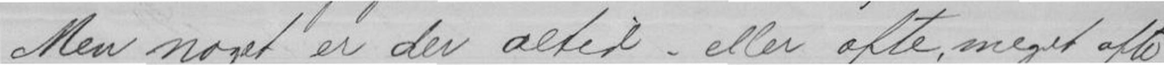Men noget er der altid - eller ofte, meget ofte
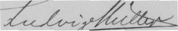Ludvig Müller
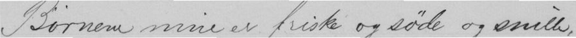Børnene mine er friske og søde og snille,
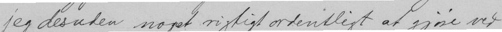jeg desuden noget rigtigt ordentligt at gjøre ved
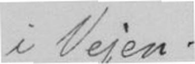i Vejen.
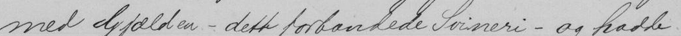med Gjælden - dette forbandede Svineri - og hadde
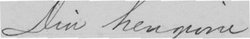Din hengivne
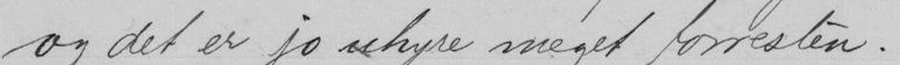og det er jo uhyre meget forresten.
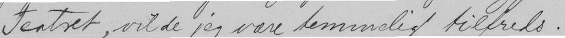Teatret, vilde jeg være temmelig tilfreds.
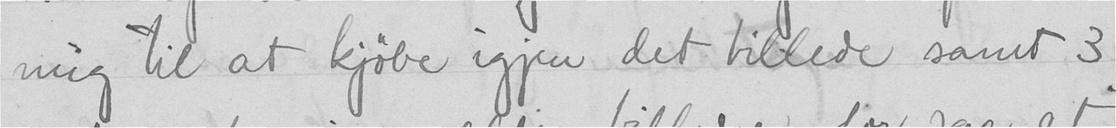mig til at kjøbe igjen det billede samt 3
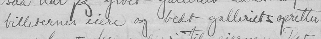billedernes eiere og bedt galleriets opretter
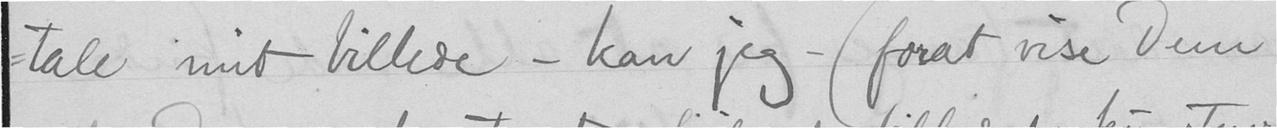tale mit billede - kan jeg (forat vise Dem
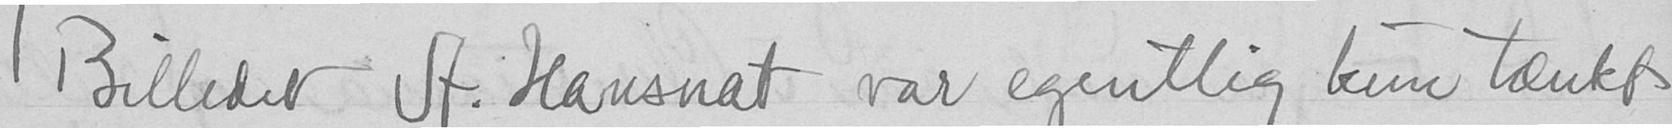Billedet St. Hansnat var egentlig kun tænkt
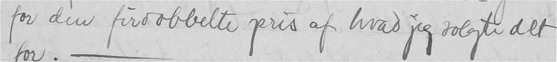for den fordobbelte pris af hvad jeg solgte det
